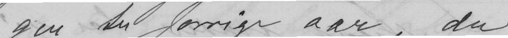gen til forrige aar, da
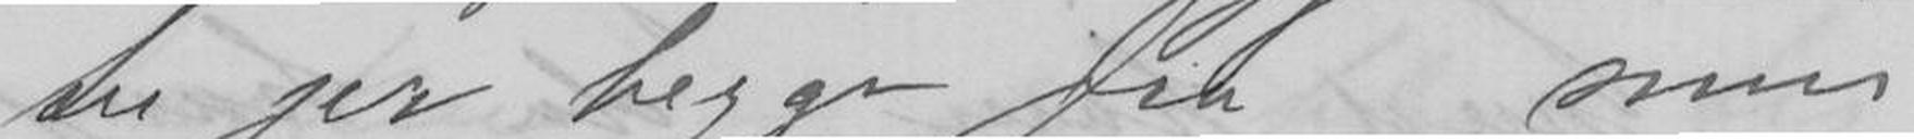til jer begge fra min
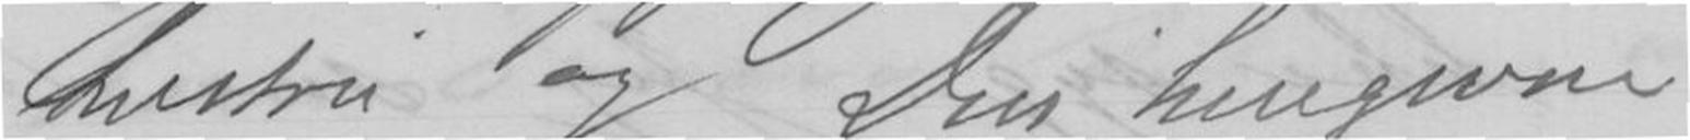hustru og Din hengivne
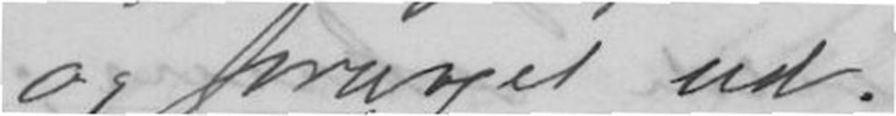og fornyet ud.
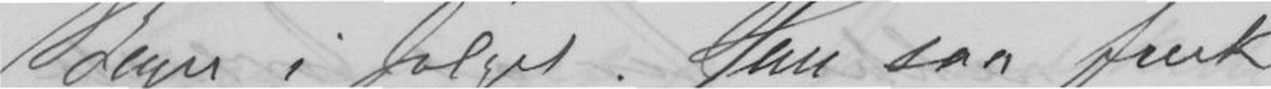Beyer i følge. Han saa frisk
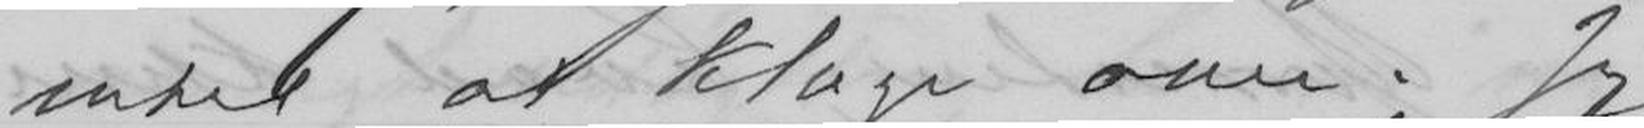intet at klage over; Jeg
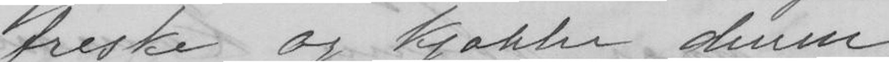friske og kjække denne
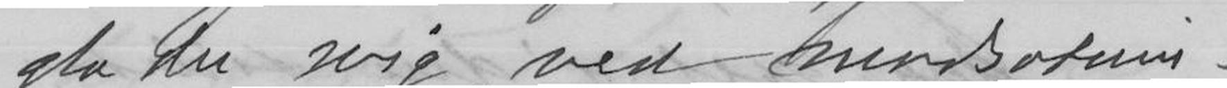glæder mig ved modsætnin-
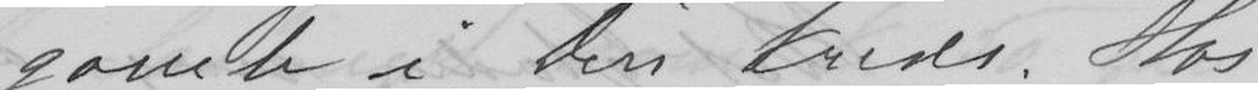gamle i Din kreds. Hos
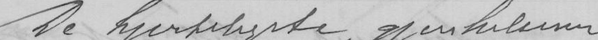De hjerteligste gjenhilsener
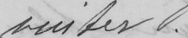vinter.
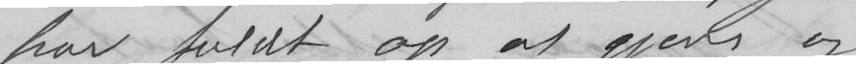har fuldt op at gjøre og
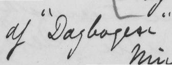af "Dagbogen"
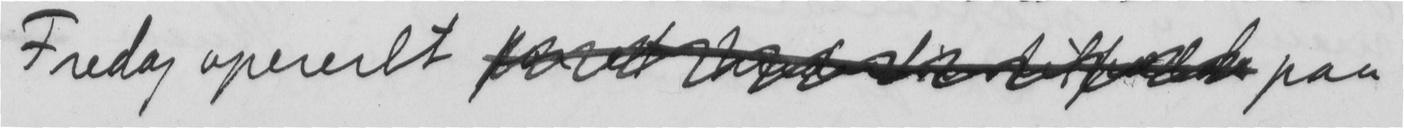Fredag opereret  paa
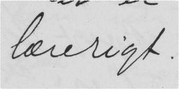lærerigt.
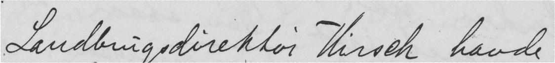Landbrugsdirektør Hirsch havde
